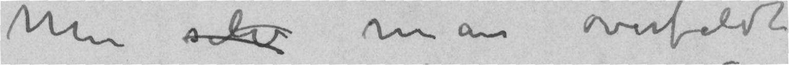Men selv man overfaldt
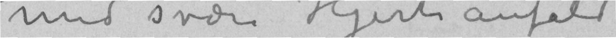med svære Hjerteanfald
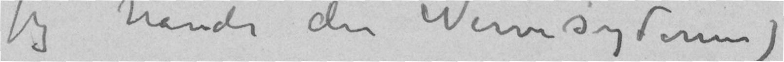jeg havde den Nervesygdom)
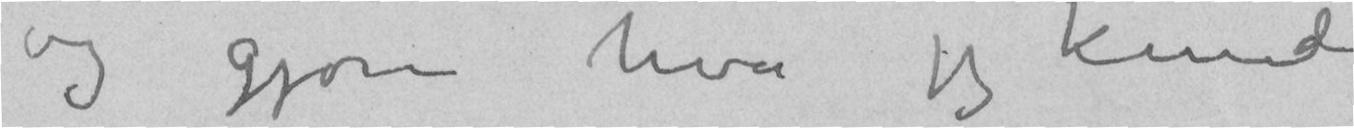og gjore hva jeg kunde
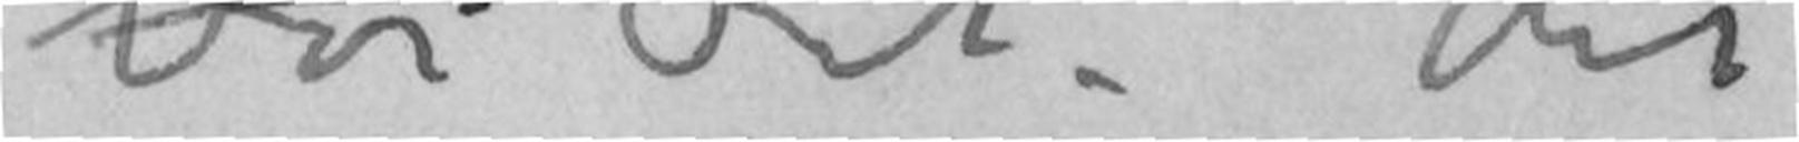vor Ret – der
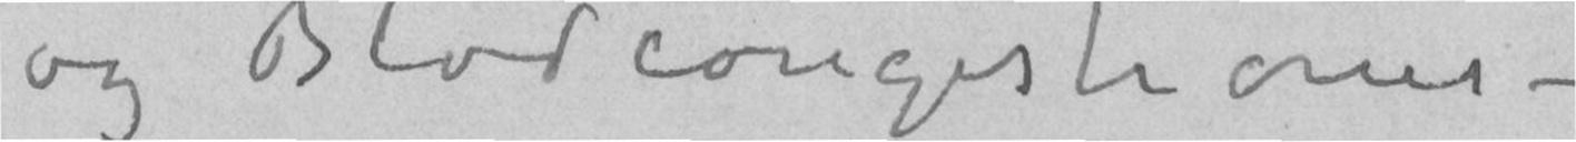og Blodcongestioner –
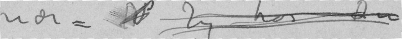nær –Jeg tror Du
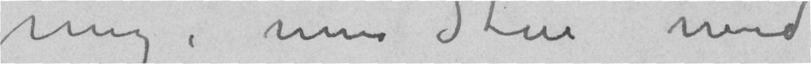mig i min Stue med
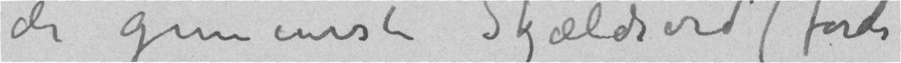de gemeneste Skjældsord (fordi
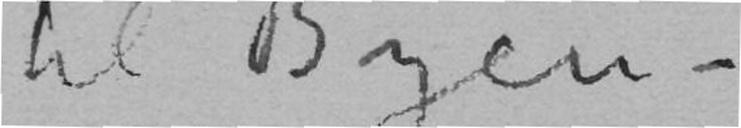til Byen –
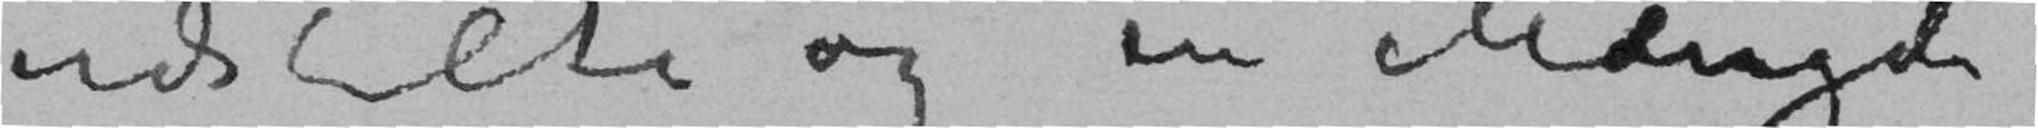udstilte og en Mængde
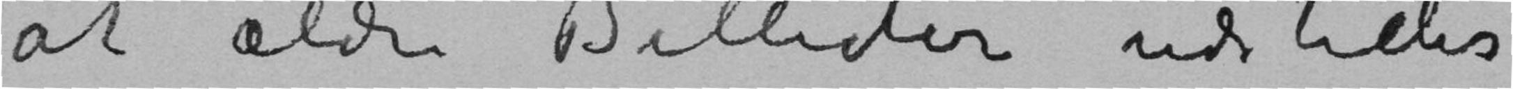at ældre Billeder udstilles
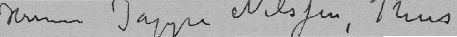Herrene Jappe Nilssen, Thiis
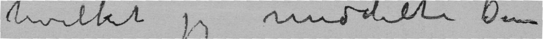hvilket jeg meddelte Dem
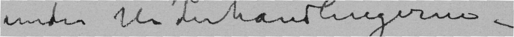under Underhandlingerne –
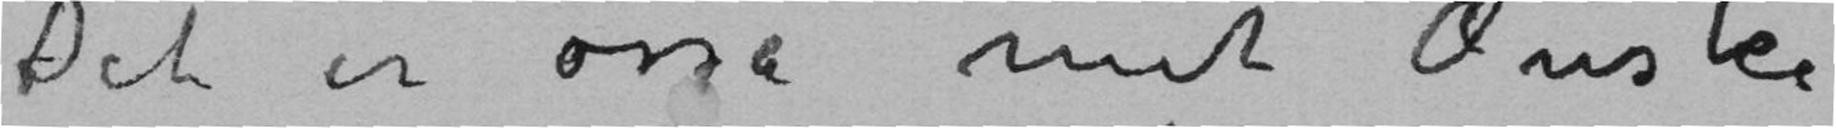Det er osså mit Ønske
